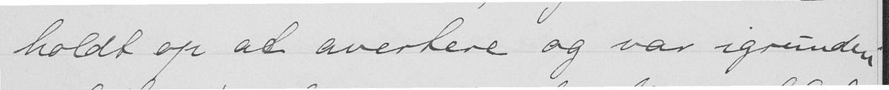holdt op at avertere og var igrunden
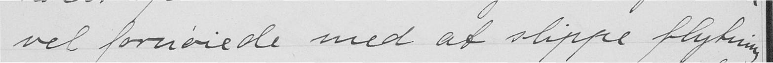vel fornøiede med at slippe flytning
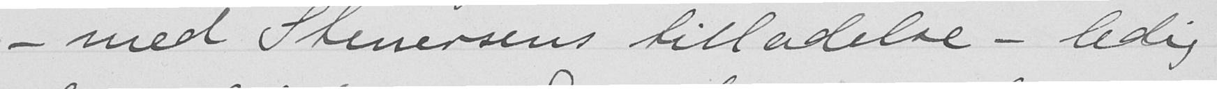- med Stenersens tilladelse - ledig
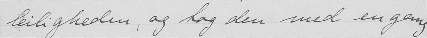leiligheden, og tog den med en gang.
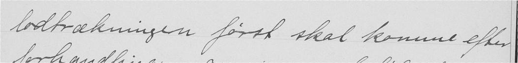lodtrækningen først skal komme efter
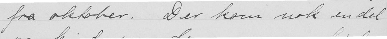fra oktober. Der kom nok en del
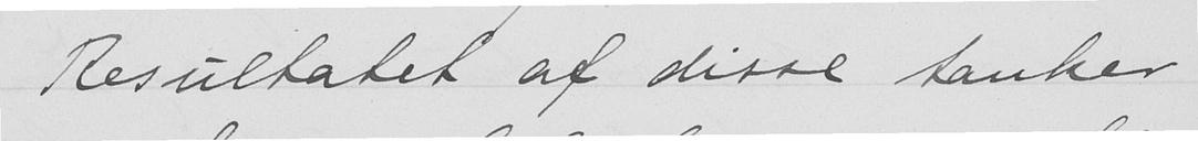Resultatet af disse tanker
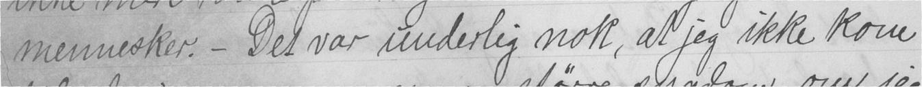mennesker. - Det var underlig nok, at jeg ikke kom
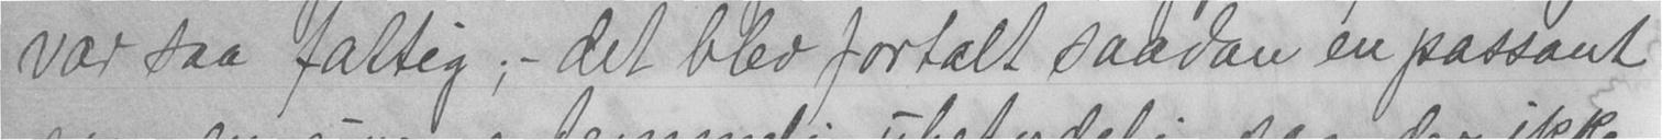var saa fattig; - det blev fortalt saadan en passant
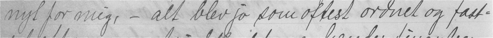nyt for mig, - alt blev jo som oftest ordnet og fast-
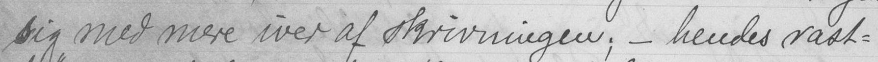sig med mere iver af skrivningen; - hendes rast-
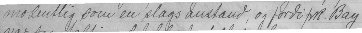modentlig som en slags anstand, og fordi frk. Bay
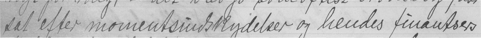sat efter momentsindskydelser og hendes finantsers
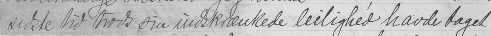sidste tid trods sin indskrænkede leilighed havde taget
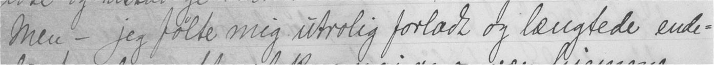Men - jeg følte mig utrolig forladt og længtede ende-
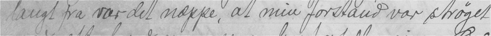langt fra var det næppe, at min forstand var strøget
